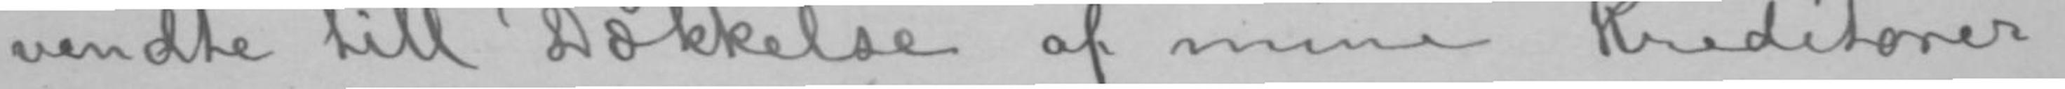vendte till Dækkelse af mine Kreditorer.
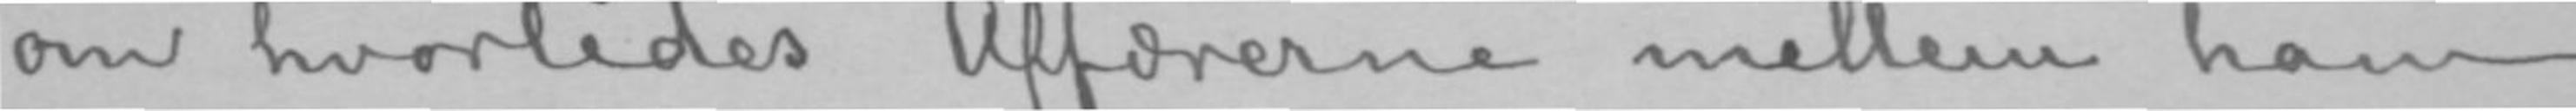om hvorledes Affærerne mellem ham
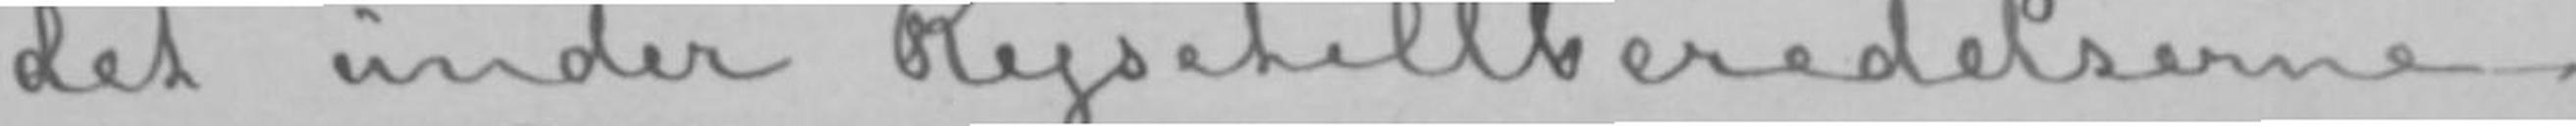det under Rejsetillberedelserne.
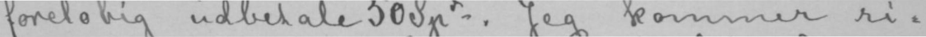foreløbig udbetale 50 Spd. Jeg kommer ri-
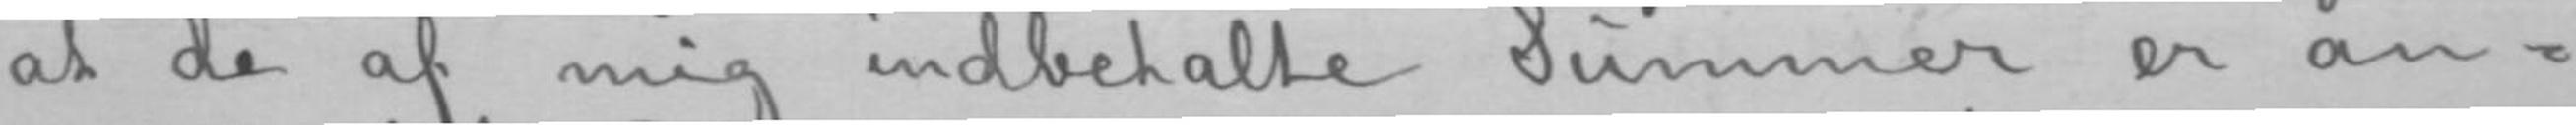at de af mig indbetalte Summer er an-
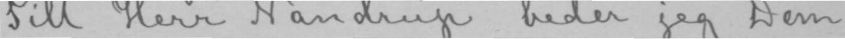Till Herr Nandrup beder jeg Dem
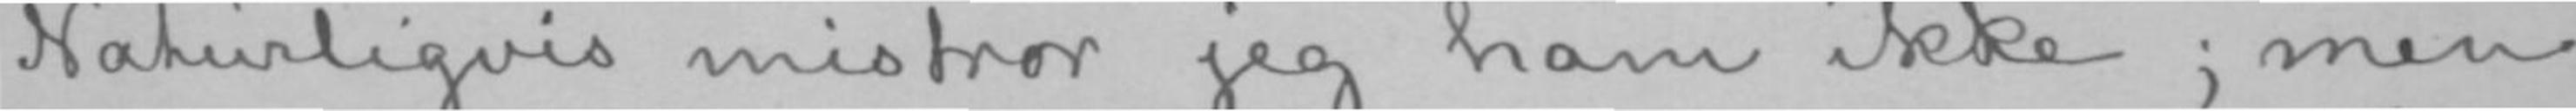Naturligvis mistror jeg ham ikke; men
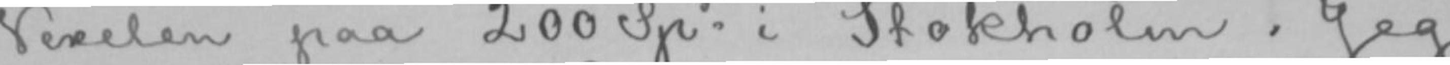Vexelen paa 200 Spd i Stokholm. Jeg
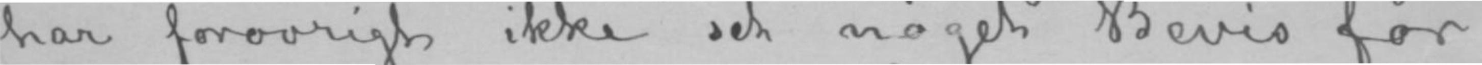har forøvrigt ikke set noget Bevis for
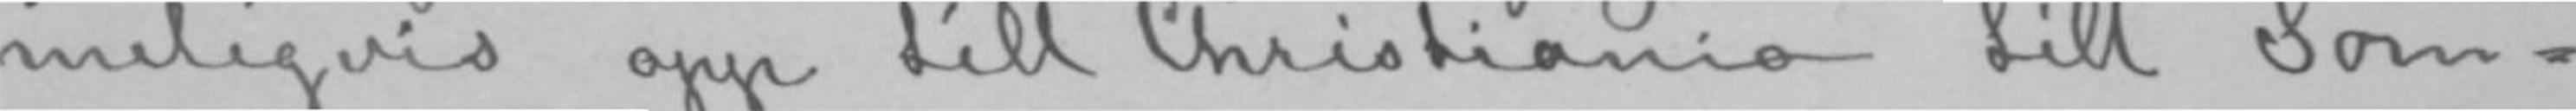meligvis opp till Christiania till Som-
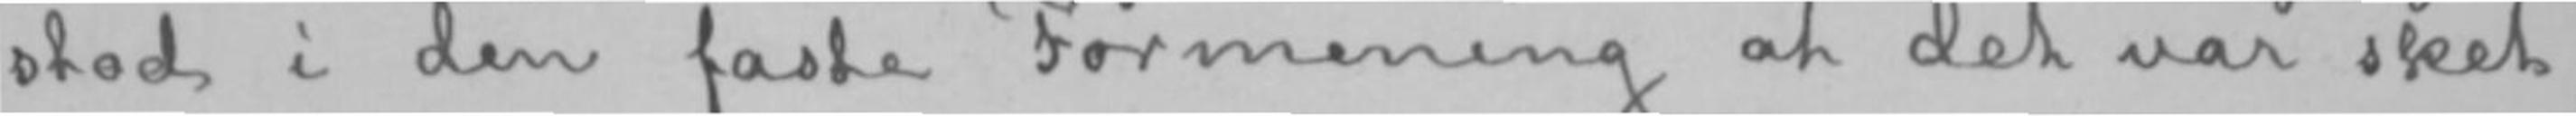stod i den faste Formening at det var sket,
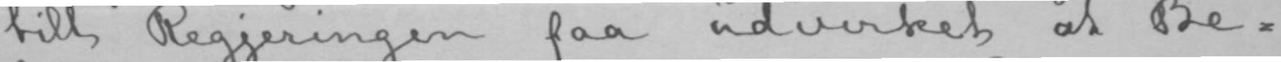till Regjeringen faa udvirket at Be-
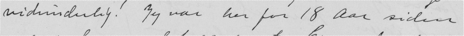vidunderlig! Jeg var her for 18 Aar siden
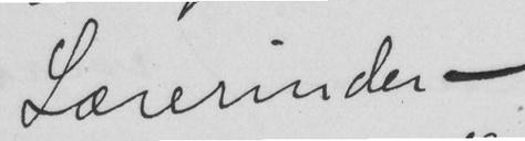Lærerinder -
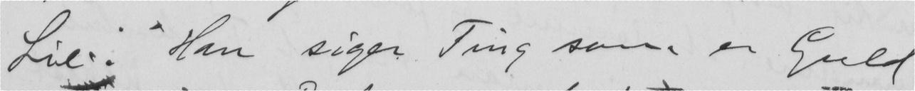Lie. Han siger Ting som er Guld
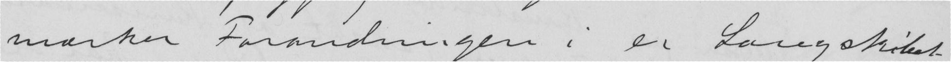mærker Forandringen i er Lang skibet
